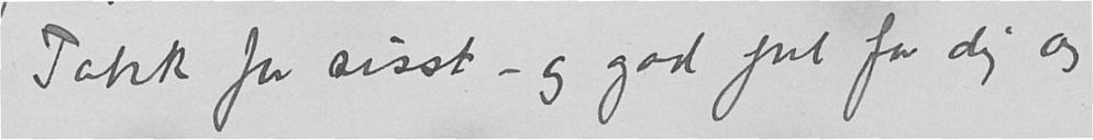Takk for sisst – og god jul for dig og
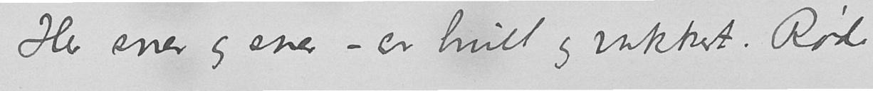Her sner og sner – er hvitt og vakkert. Røde
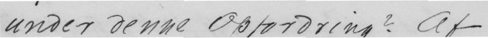under denne Opfordring? Af
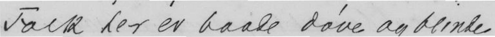Folk her er baade døve og blinde
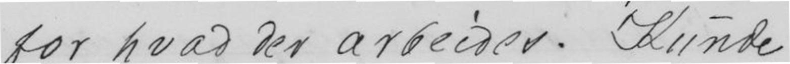for hvad der arbeides. Kunde
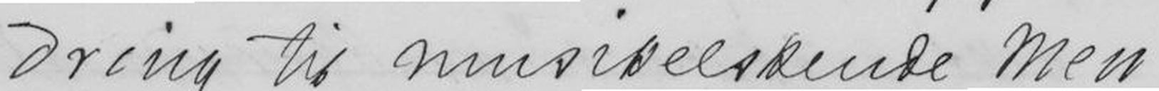dring til Musikelskende Men-
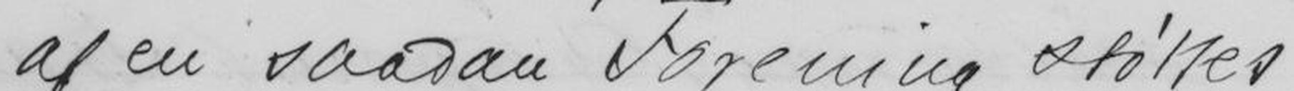af en saadan Forening støttes
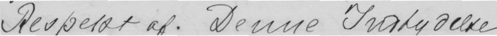Respekt af. Denne Indbydelse
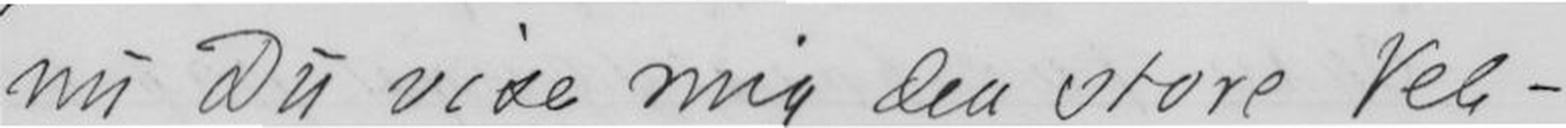nu Du vise mig den store Vel-
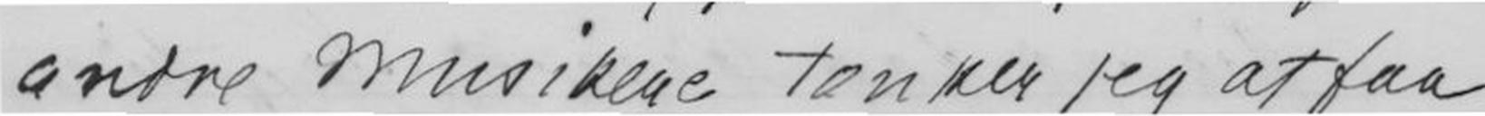andre musikere tenker jeg at faa
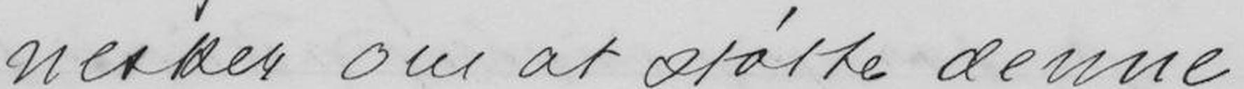nesker om at støtte denne
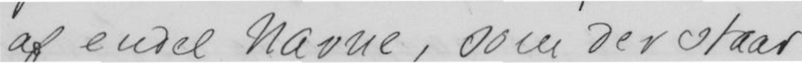af endel Navne, som der staar
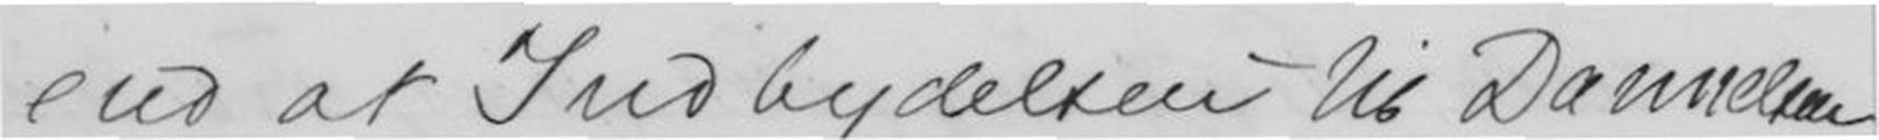end at Indbydelsen til Dannelsen
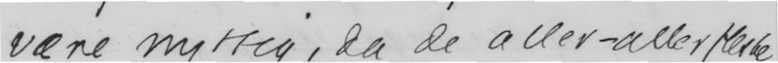være nyttig, da de aller-aller fleste
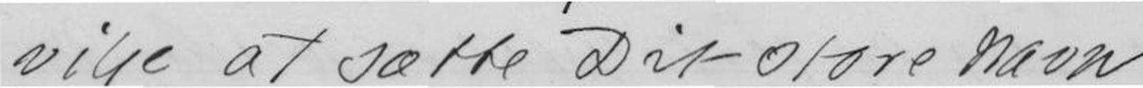vilje at sætte Dit store navn
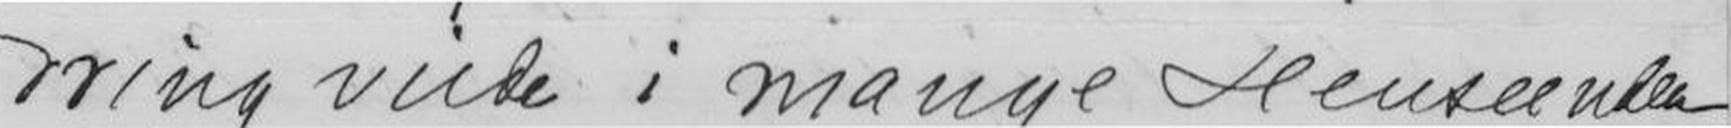dring vilde i mange Henseende
